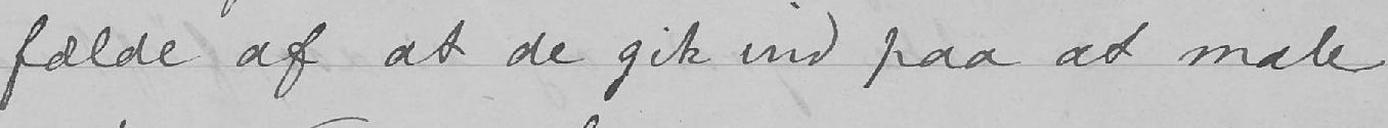fælde af at de gik ind paa at male
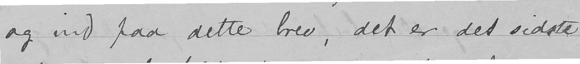og ind paa dette brev, det er det sidste
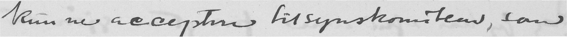kunne acceptere tilsynskomiteen, som
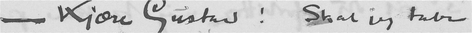- Kjære Gustav! Skal jeg tabe
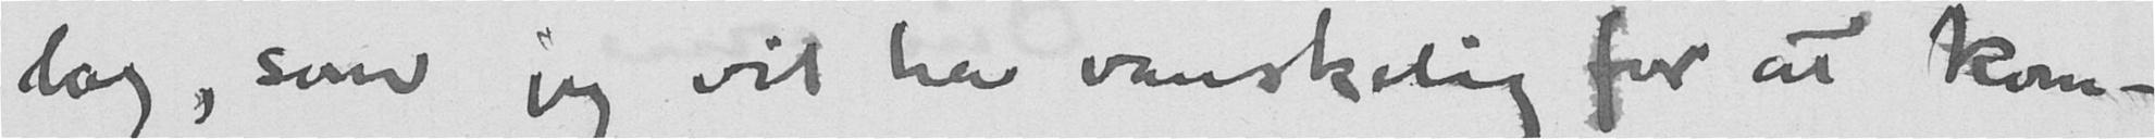lag, som jeg vil ha vanskelig for at kom-
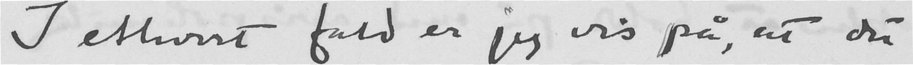I ethvert fald er jeg vis på, at du
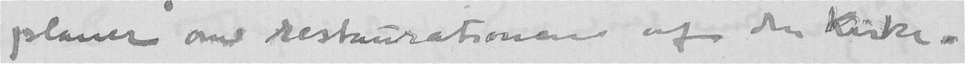planer on restaurationen af den kirke
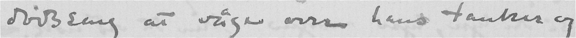dødsseng at våge over hans tanker og
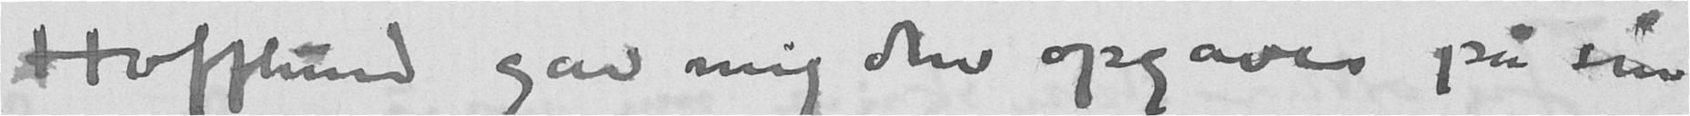Hofflund gav mig den opgave på sin
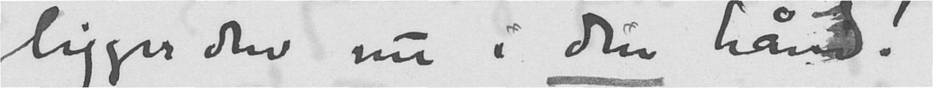ligger den nu i din hånd!
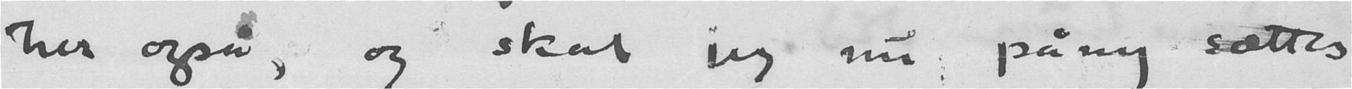her også, og skal jeg nu påny sættes
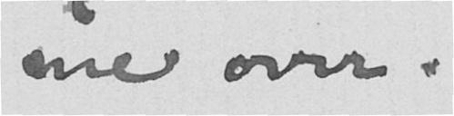me over.
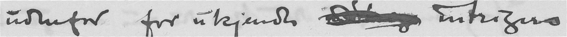udenfor for ukjende intrigers
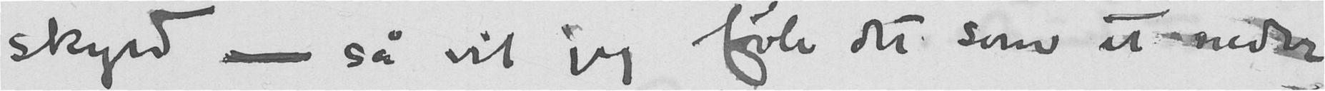skyld - så vil jeg føle det som et neder-
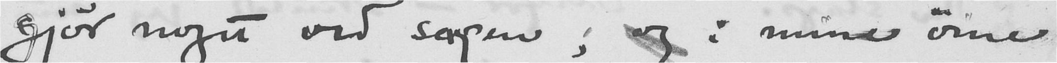gjør noget ved sagen; og i mine øine
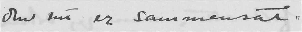den nu er sammensat.
